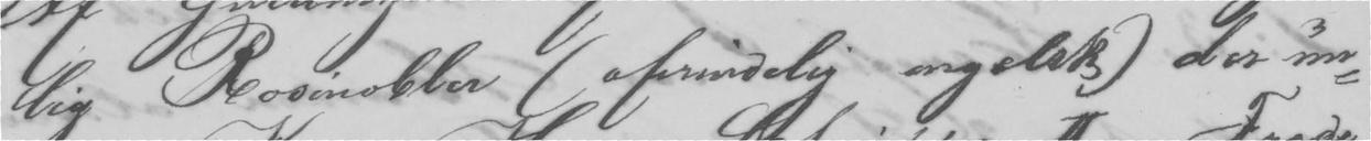lig Rosinobler (oprindelig engelsk) der un-
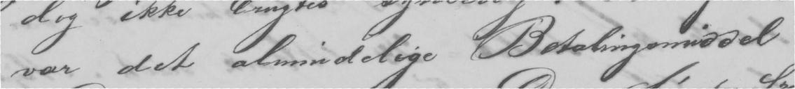var det almidelige Betalingsmiddel
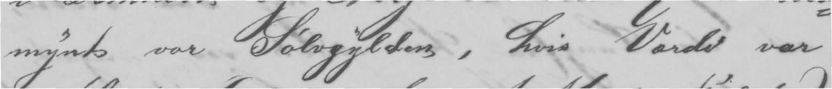mynt var Sølvgylden, hvis Værdi var
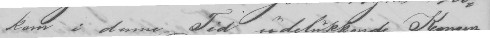kom i denne Tid udelukkende Kongen,
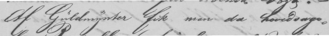Af Guldmynter fik man da hovedsage-
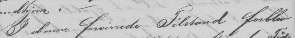I denne forvirrede Tilstand forblev
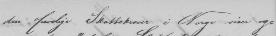den ferdige Skattekronen i Norge viser og-
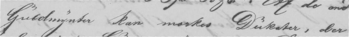Guldmynter kan mærkes Dukater, der
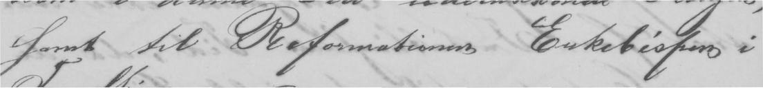samt til Reformationen Erkebispen i
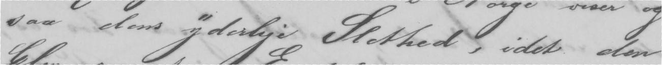saa dens yderlige Slethed, idet den
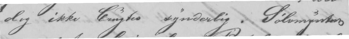dog ikke brugtes synderlig: Sølvmynten
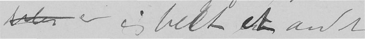blev er jeg helt et andet
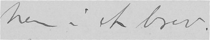hen i et brev!
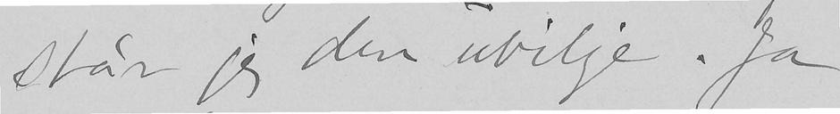står jeg den uvilje. Ja
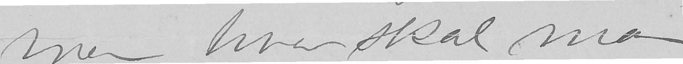men hvor skal man
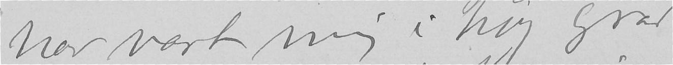har vært mig i høj grad
